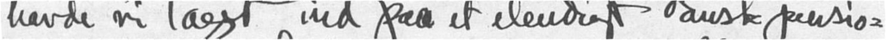havde vi taget ind paa et elendigt dansk pensio-
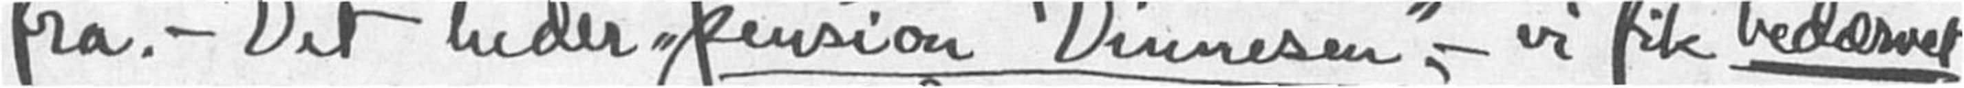fra. - Det heder "Pension Dinnesen", - vi fik bedærvet
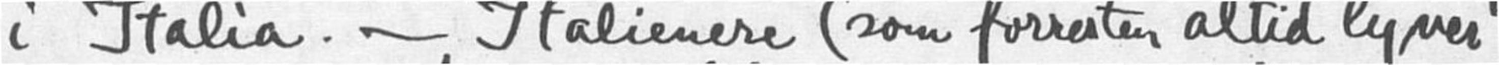i Italia. - Italienerne (som forresten altid lyver)
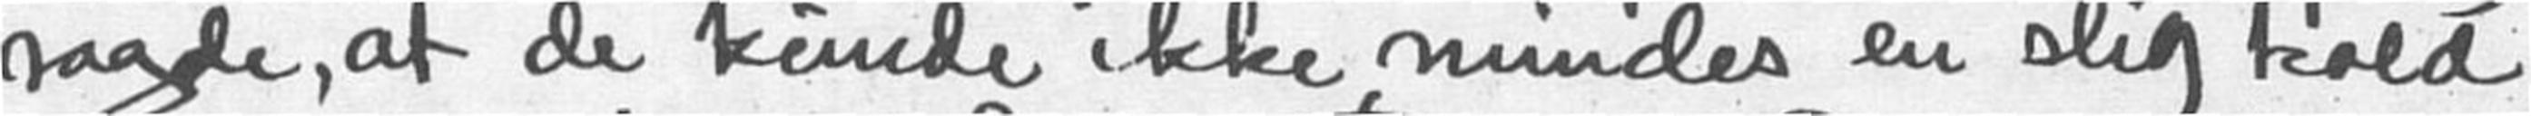sagde, at de kunde ikke mindes en slik kold
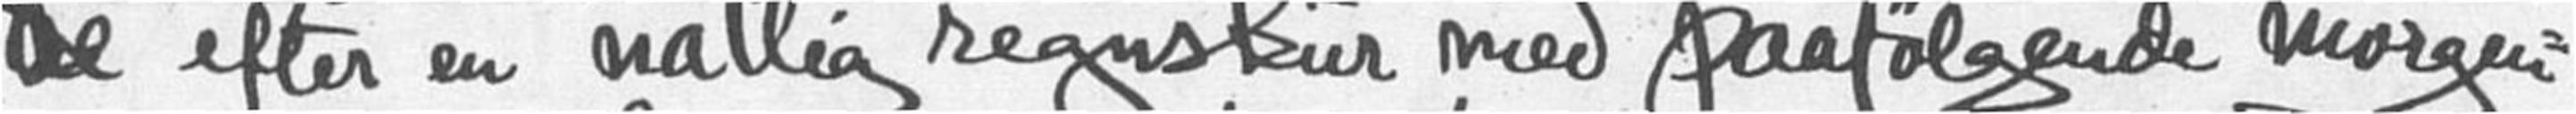de efter en natlig regnskur med paafølgende morgen-
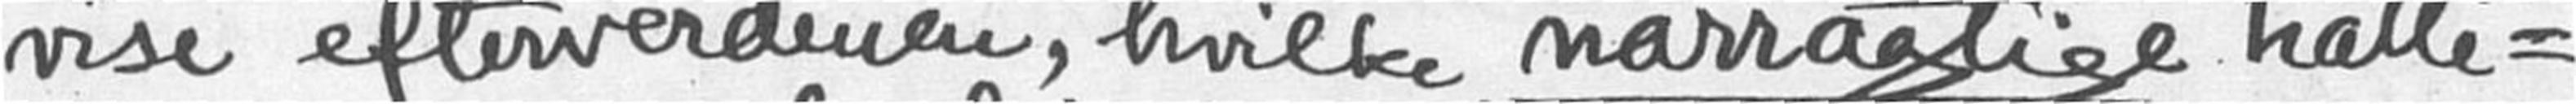vise efterverdenen, hvilke narragtige hatte-
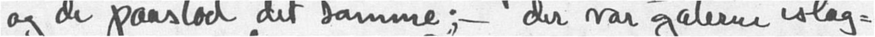og de paastod det samme; - der var gaterne islag-
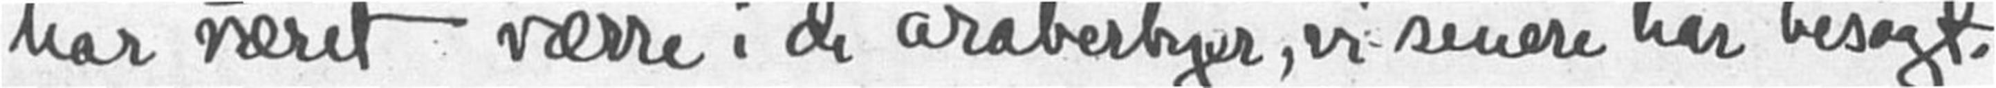har været værre i de araberbyer, vi senere har besøgt.
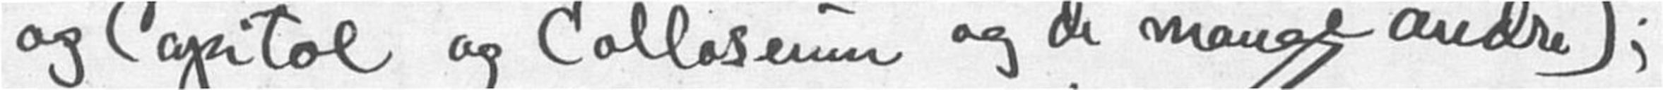og Capitol og Colloseum og de mange andre);
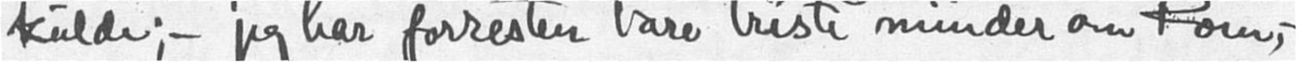kulde; - jeg har forresten bare triste minder om Rom
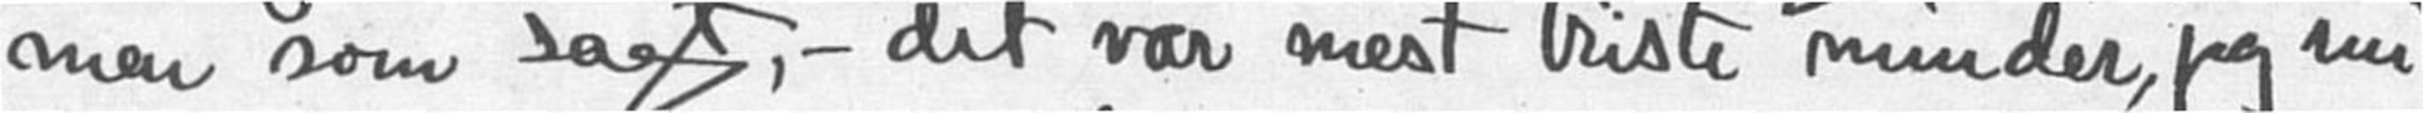men som sagt, - det var mest triste minder, jeg nu
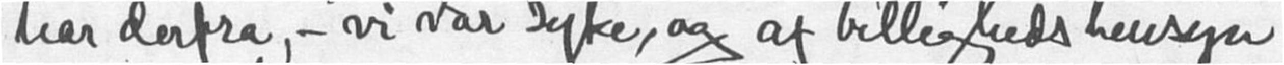har derfra,- vi var syke, og af billighedshensyn
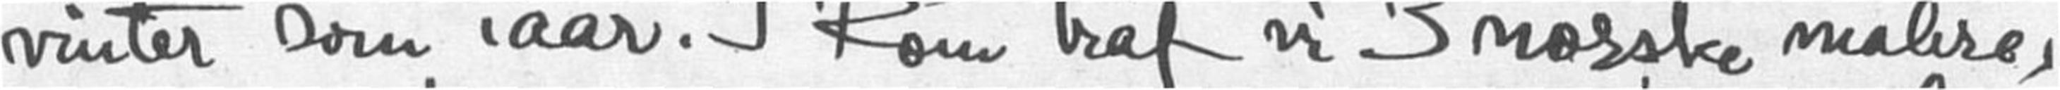vinter som iaar. I Rom traf vi 3 norske malere,
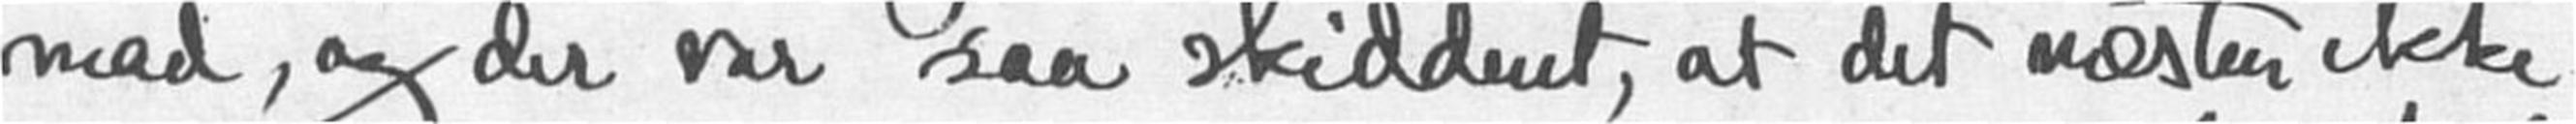mad, og der var saa skiddent, at det næsten ikke
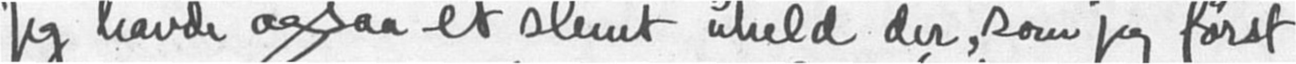Jeg havde ogsaa et slemt uheld der, som jeg først
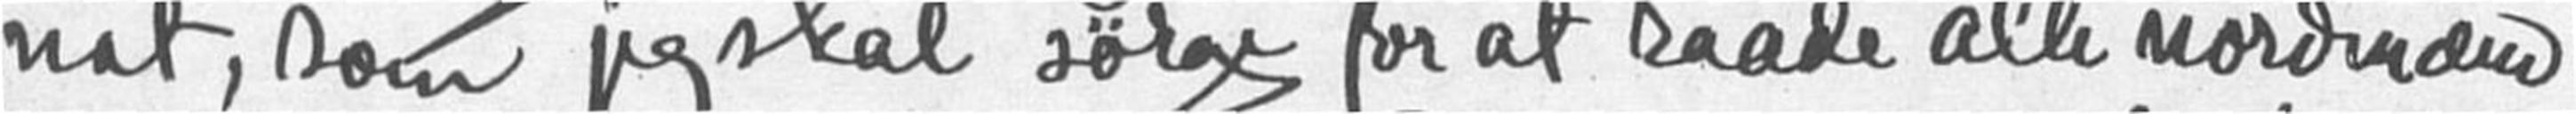nat, som jeg skal sørge for at raade alle nordmænd
